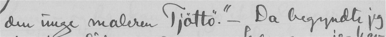den unge maleren Tjøttø. - Da begyndte jeg
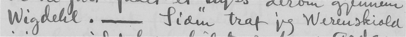Wigdehl. - Siden traf jeg Werenskiold
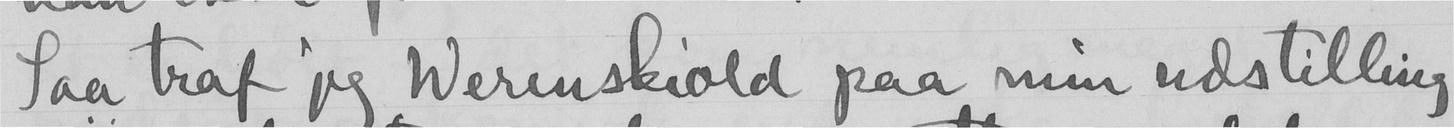Saa traf jeg Werenskiold paa min udstilling
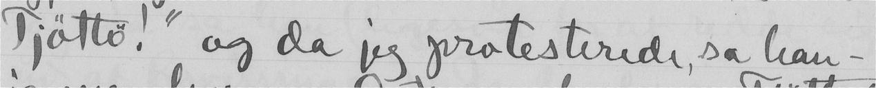Tjøttø!" og da jeg protesterede, sa han -
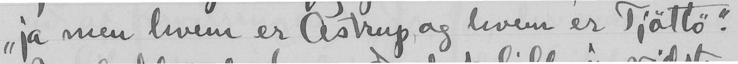"ja men hvem er Astrup, og hvem er Tjøttø".
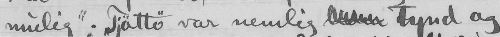mulig". Tjøttø var nemlig  tynd og
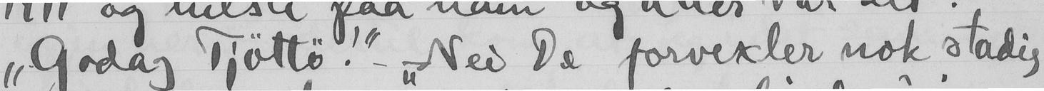"Goddag Tjøttø!" -"Nei De forvexler nok stadig
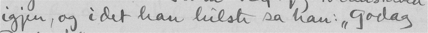igjen, og i det han hilste sa han: "godag
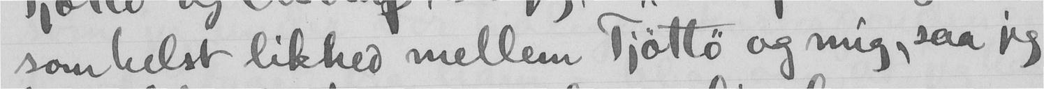som helst likhed mellem Tjøttø og mig, saa jeg
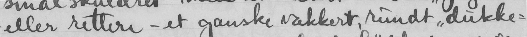eller rettere - et ganske vakkert, rundt "dukke-
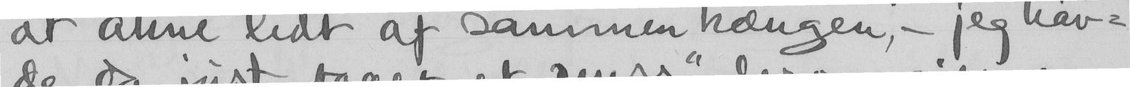at ahne lidt af sammen hængen, - jeg hav-
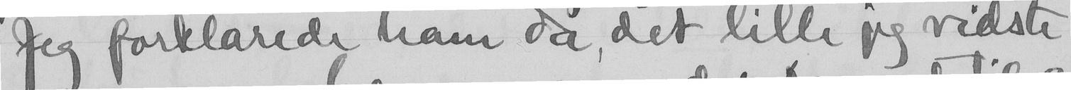Jeg forklarede ham da, det lille jeg vidste
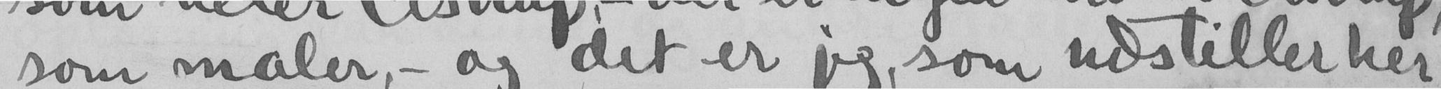som maler, - og det er jeg, som udstiller her
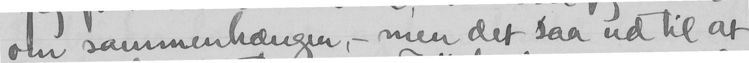om sammenhængen, - men det saa ud til at
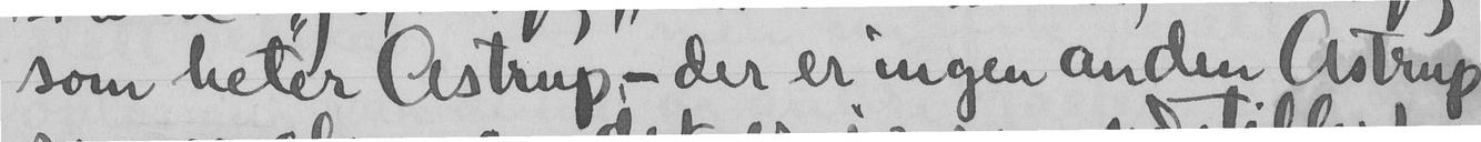som heter Astrup,- der er ingen anden Astrup
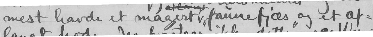mest havde et magert "faunefjæs" og et af-
